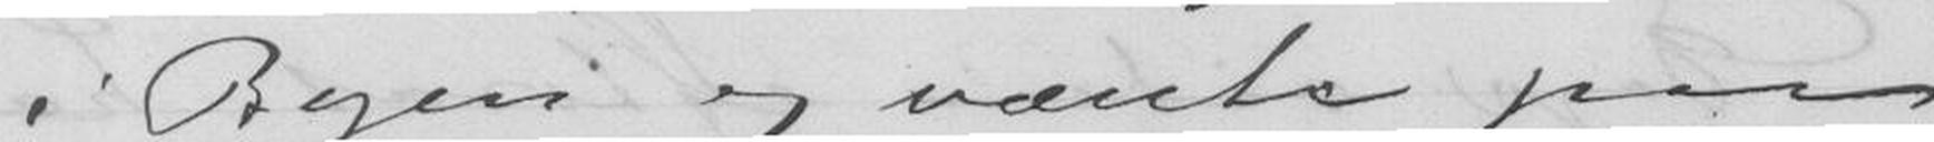i Byen og vænte paa
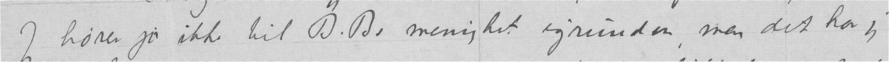Jeg hører jo ikke til B.Bs menighet igrunden, men det har jeg
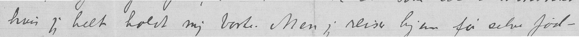hvis jeg helt holdt mig borte. Men jeg reiser hjem før selve fød-
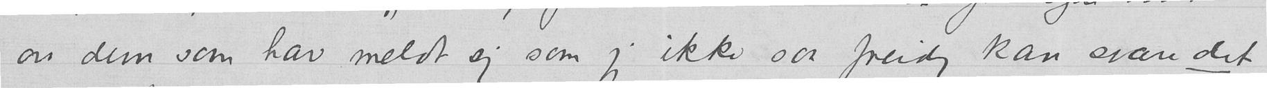av dem som har meldt sig som jeg ikke saa freidig kan svare det
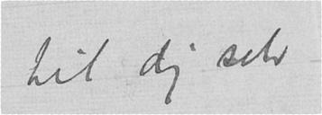til dig selv
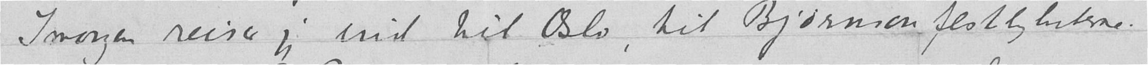Imorgen reiser jeg ind til Oslo, til Bjørnsonfestligheterne.
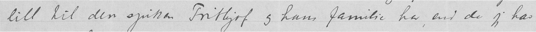litt til den sjuken Frithjof og hans familie har, end de jeg har
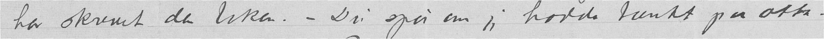har skrevet den boken. – Du spør om jeg hadde tænkt paa otta-
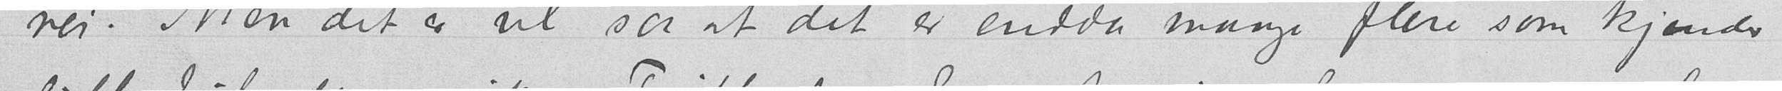nei. Men det er vel saa at det er endda mange flere som kjender
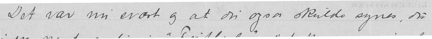Det var nu svært og at du ogsaa skulde synes, du
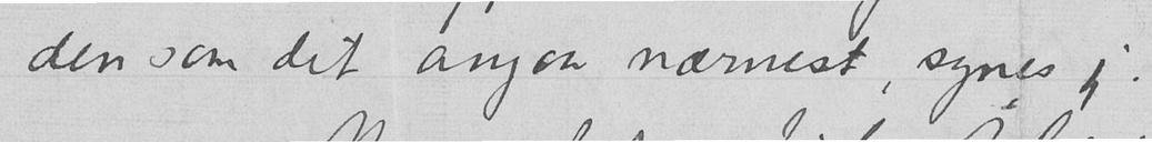den som det angaar nærmest, synes jeg.
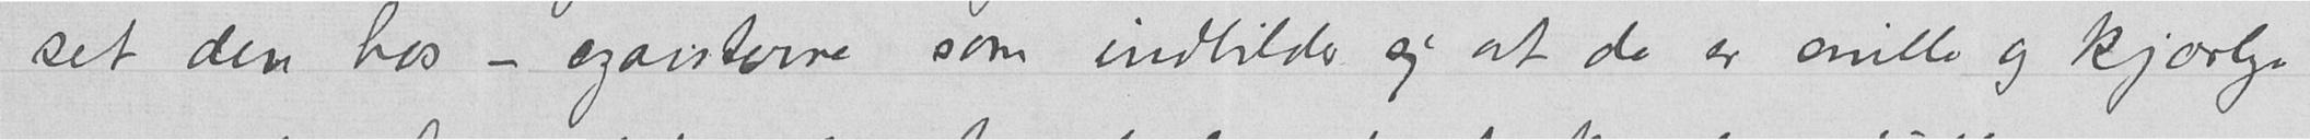set den hos – egoisterne som indbilder sig at de er snille og kjærlige
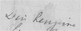Din hengivne
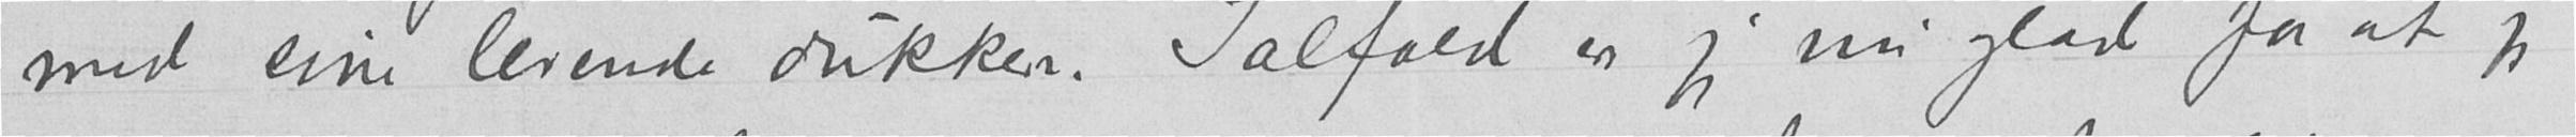med sine levende dukker. Ialfald er jeg nu glad for at jeg
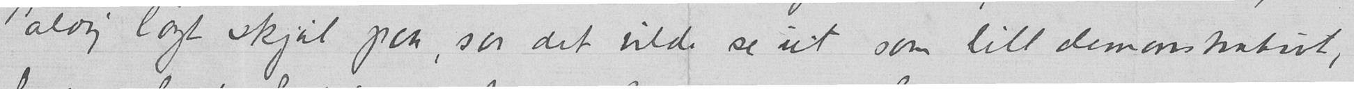aldrig lagt skjul paa, saa det vilde se ut som litt demonstrativt,
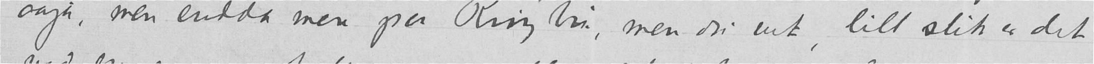aaja, men endda mere paa Ringebu, men du vet, litt slik er det
